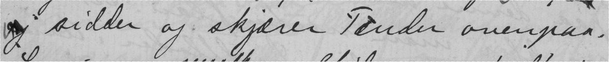sidder og skjærer Tænder ovenpaa.
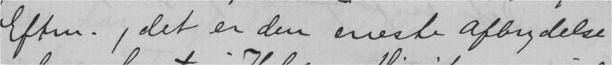Efterm., det er den eneste Afbrydelse
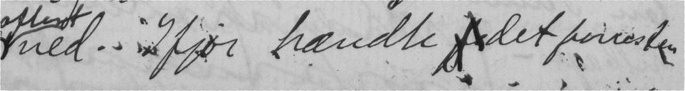ned. Ifjor hændte det forresten
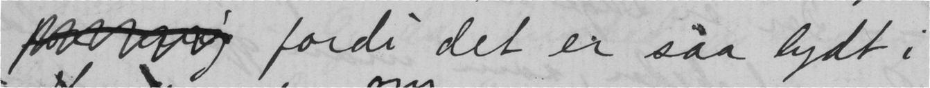fordi det er saa lydt i
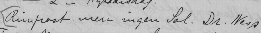Rimfrost men ingen Sol. Dr. Ness
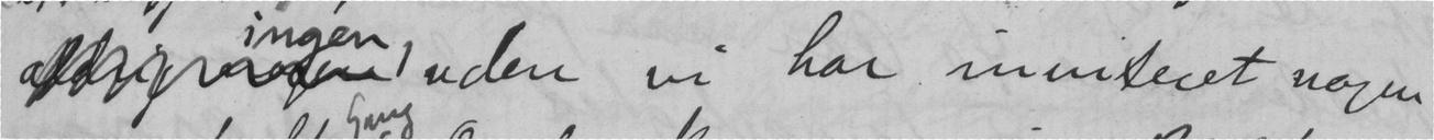uden vi har inviteret nogen
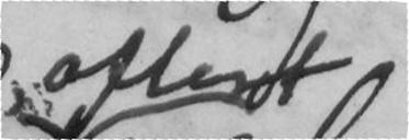oftest
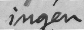ingen
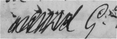G.
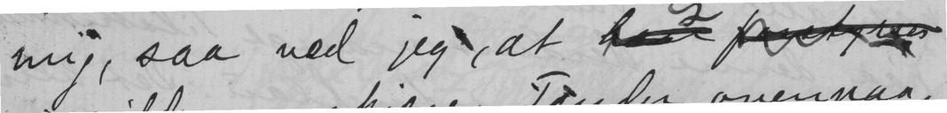mig, saa ved jeg, at
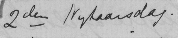2de Nytaarsdag.
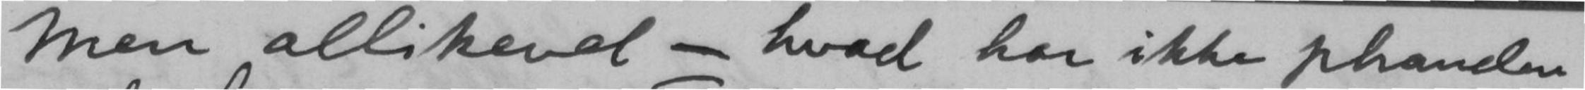Men allikevel - hvad har ikke phanden
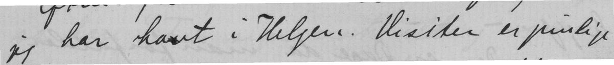jeg har havt i Helgen. Vi siter er pinlige
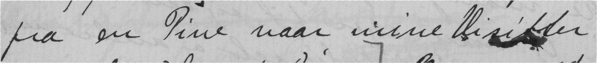fra en Pine naar mine Visitter
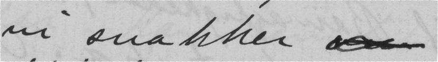vi snakker
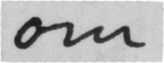om
